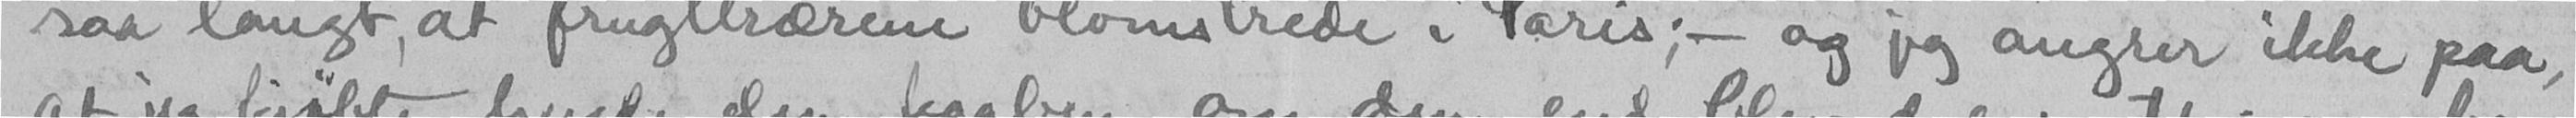saa langt, at frugttrærene blomstrede i Paris; - og jeg angrer ikke paa,
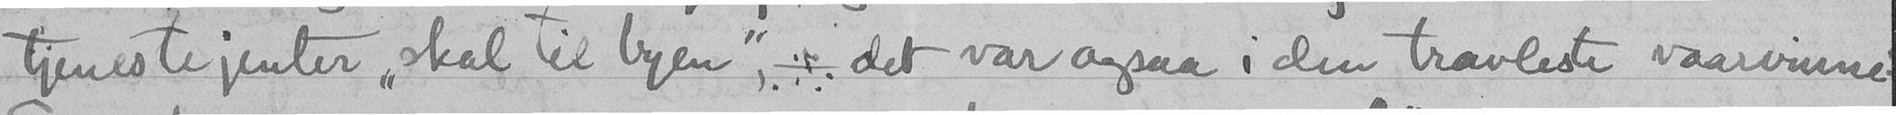tjenestejenter "skal til byen", - det var ogsaa i den travleste vaarvinne
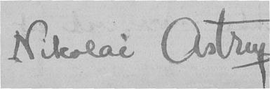Nikolai Astrup
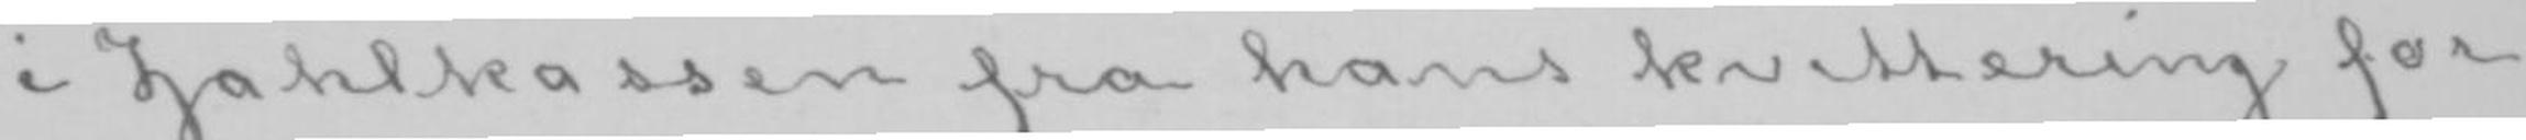i Zahlkassen fra hans kvittering for
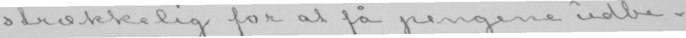strækkelig for at få pengene udbe-
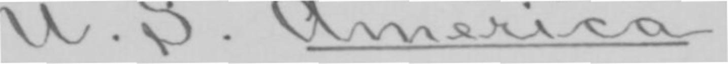U. S. America.
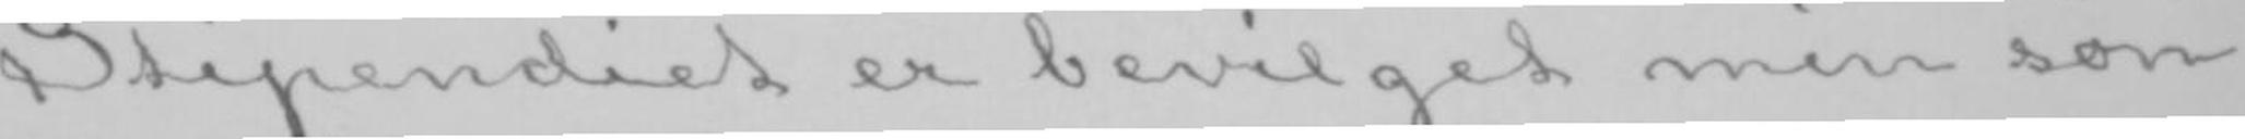Stipendiet er bevilget min søn
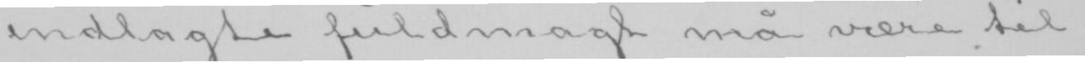indlagte fuldmagt må være til-
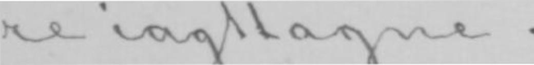re iagttagne.
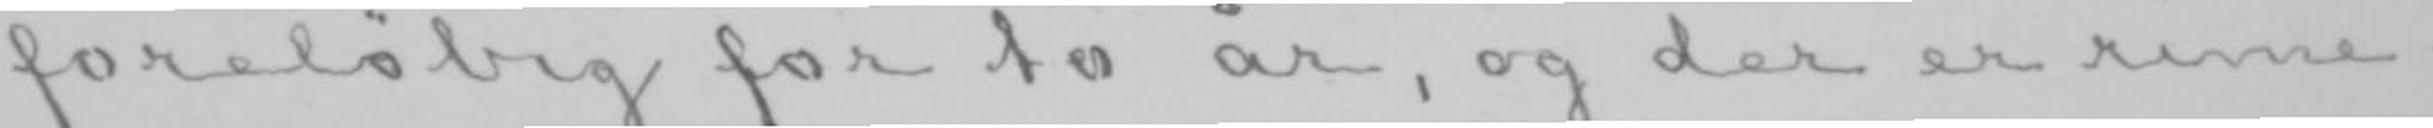foreløbig for to år, og der er rime-
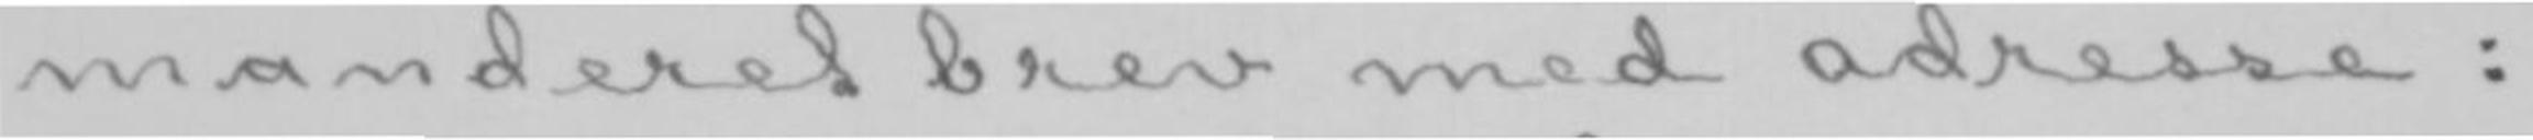manderet brev med adresse:
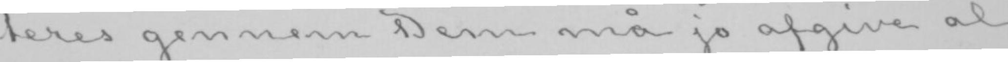teres gennem Dem må jo afgive al
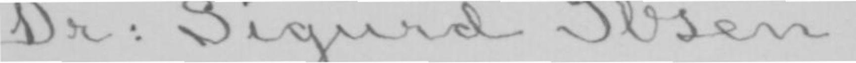Dr: Sigurd Ibsen.
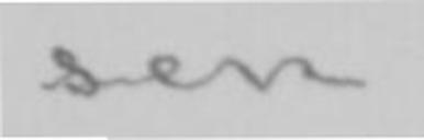sen.
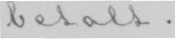betalt.
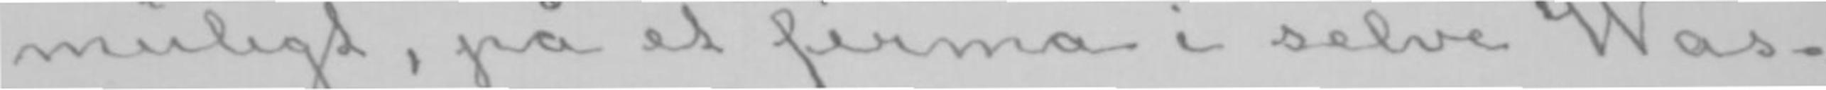muligt, på et firma i selve Was-
